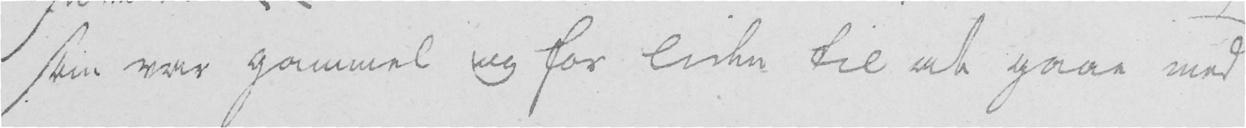som var gammel og for liden til at gaae med
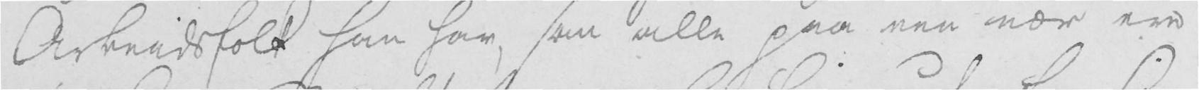Arbeidsfolk han har, som alle paa een nær ere
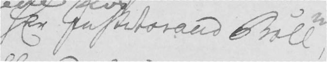Hr Justitsraad Boll,
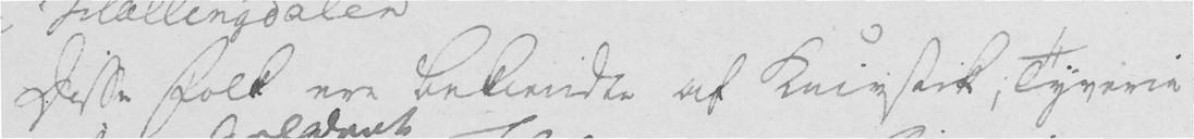Disse Folk ere bekiendte af Knivstik; Tyverie
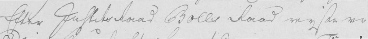Efter Justits Raad Bolls Raad reyste vi
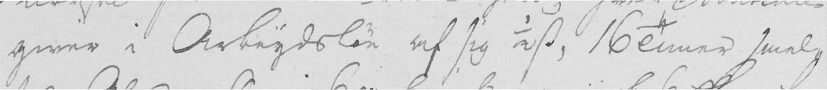giver i Arbeydsløn af sig 1/2 p, 16 Timer smel-
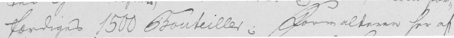færdiges 1500 Bouteiller; Forvalteren her af
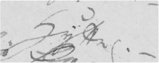Hytte. -
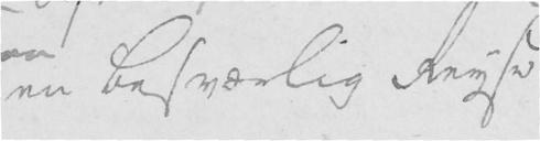en besværlig Reyse
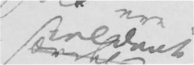sieldent
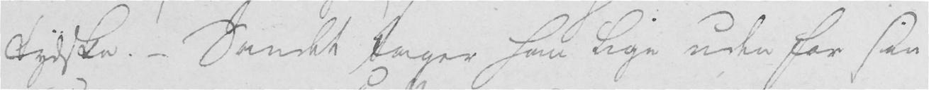tydske. - Sandet tager han lige uden for sin
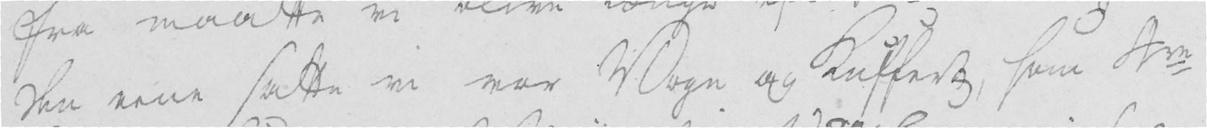den eene satte vi vor Vogn og Kuffert, som 4re
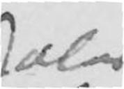aler
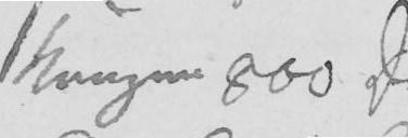Kongen 800 D
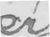er
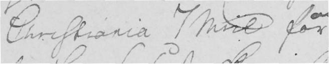Christiania 7 Miil for
